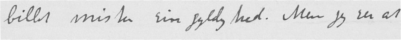billet mister sin gyldighed. Men jeg ser at
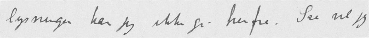lysninger kan jeg ikke gi herfra. Saa vil jeg
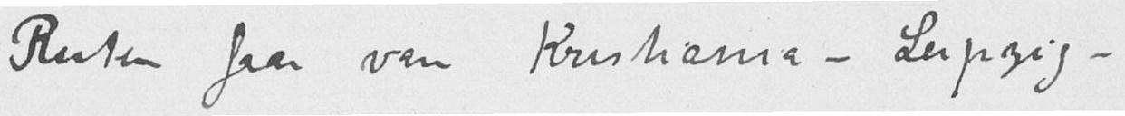Ruten faar være Kristiania-Leipzig-
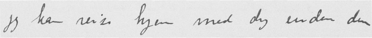jeg kan reise hjem med dig inden din
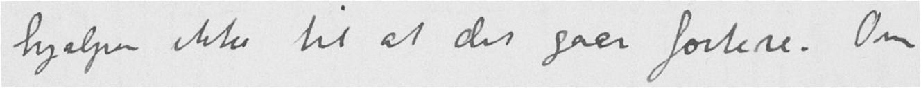hjælper ikke til at det gaar fortere. Om
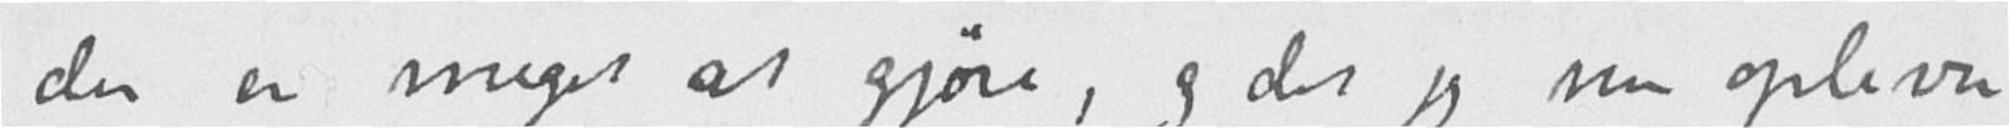der er meget at gjøre, og det jeg nu oplever
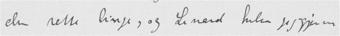den rette linje, og Lenard hilser jeg gjerne
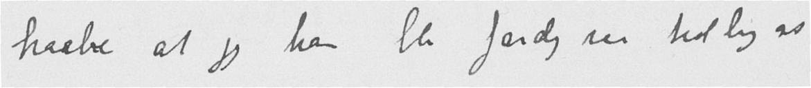haabe at jeg kan bli færdig saa tidlig at
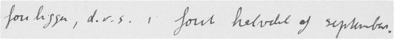foreligger, d.v.s. i første halvdel af september.
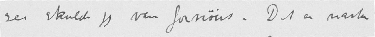saa skulde jeg være fornøiet. Det er næsten
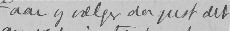aar og vælger da just det
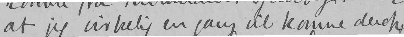at jeg virkelig en gang vil komme derop,
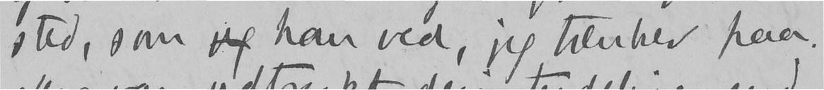sted, som jeg han ved, jeg tænker paa.
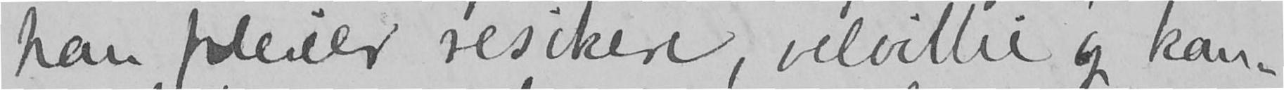han pleier resikere, velvillie og kan-
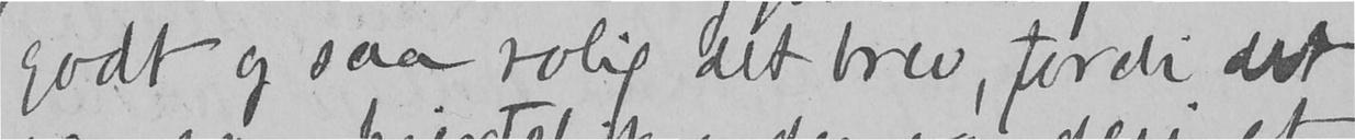godt og saa rolig det brev, fordi det
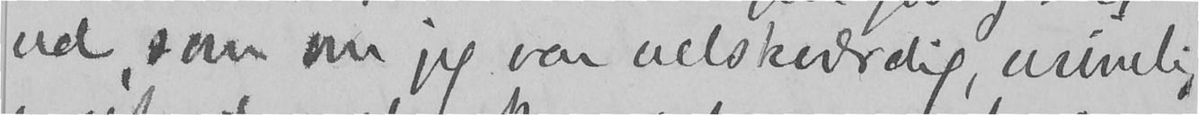ud, som om jeg var uelskværdig, urimelig
